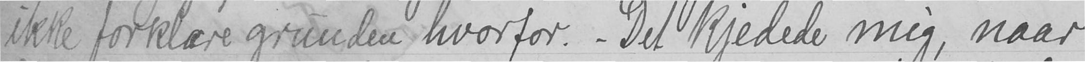ikke forklare grunden hvorfor. - Det kjedede meg, naar
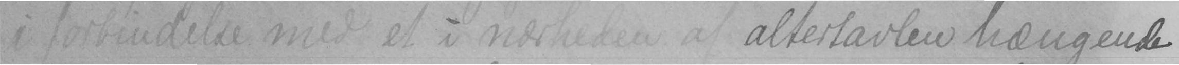i forbindelse med et i nærheden af altertavlen hængende
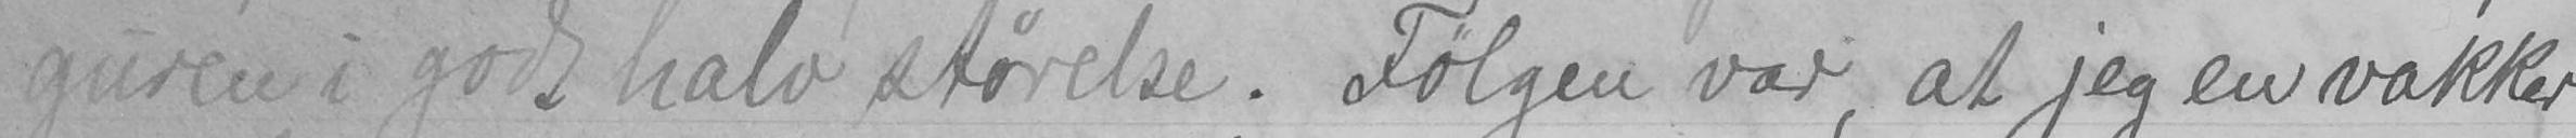guren i godt halv størelse. Følgen var, at jeg en vakker
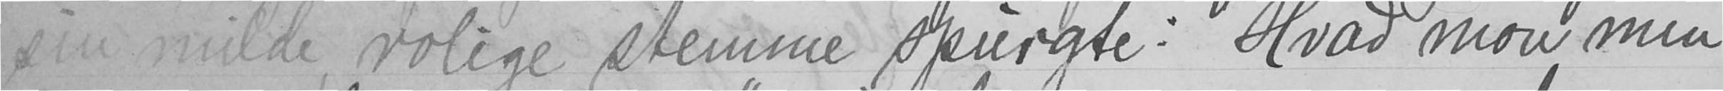sin milde, rolige stemme spurgte: "Hvad mon min
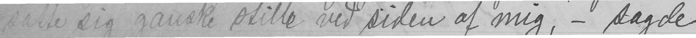satte sig ganske stille ved siden af mig, - sagde
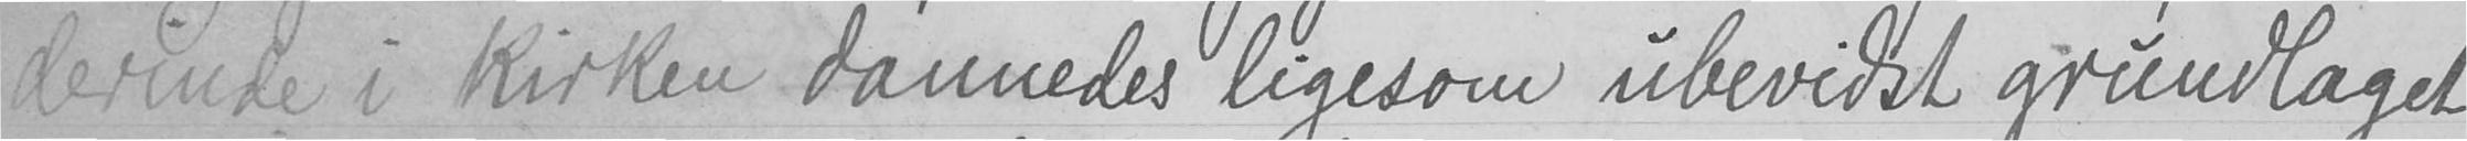derinde i kirken dannedes ligesom ubevidst grundlaget
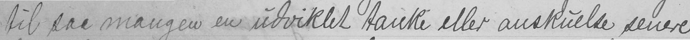til saa mangen en udviklet tanke eller anskuelse senere
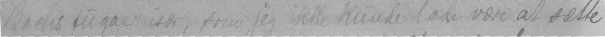Bachs fugaer især, som jeg ikke kunde lade være at sætte
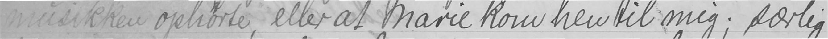musikken ophørte, eller at Marie kom hen til meg; særlig
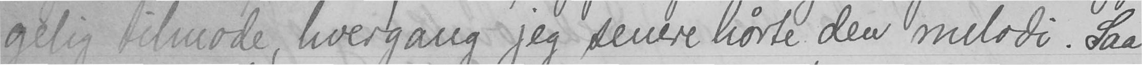gelig tilmode, hvergang jeg senere hørte den melodi. Saa
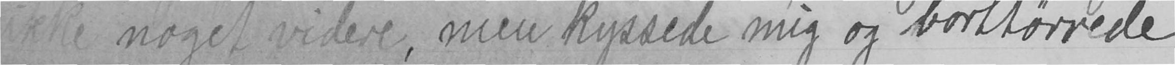ikke noget videre, men kyssede mig og borttørrede
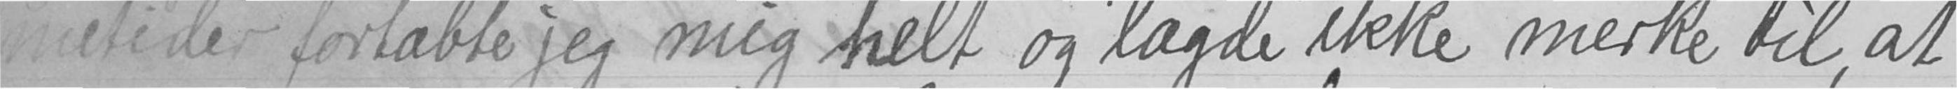metider fortabte jeg mig helt og lagde ikke merke til, at
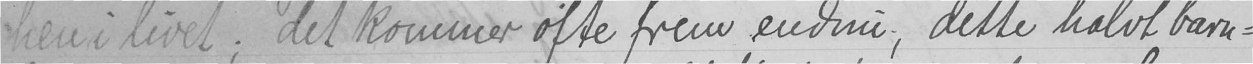her i livet; det kommer ofte frem endnu, dette halvt barn-
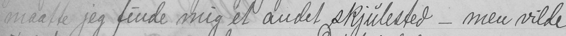maatte jeg finde mig er andet skjulested - men vilde
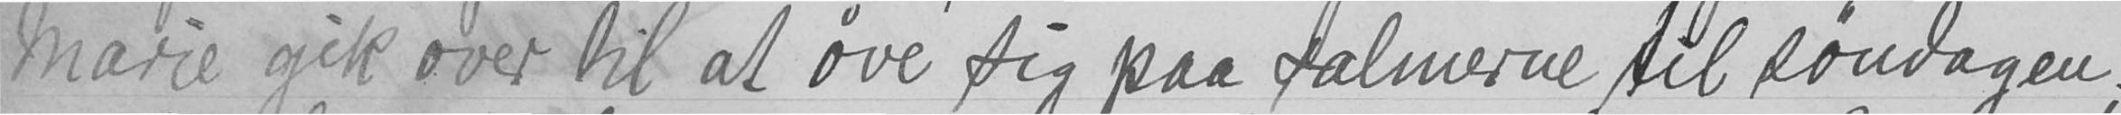Marie gik over til at øve sig paa salmerne til søndagen;
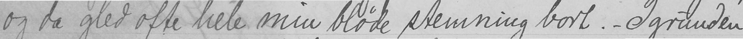og da gled ofte hele min bløde stemning bort. - Igrunden
